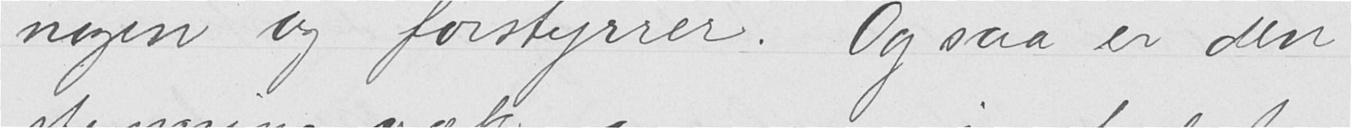nogen og forstyrrer. Og saa er den
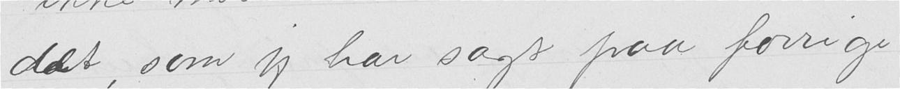det, som jeg har sagt paa forrige
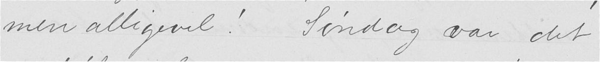men alligevel! Søndag var det
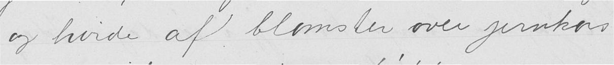og hvide af blomster over jernkors
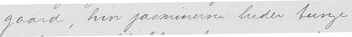gaard, hvor jasminerne luder tunge
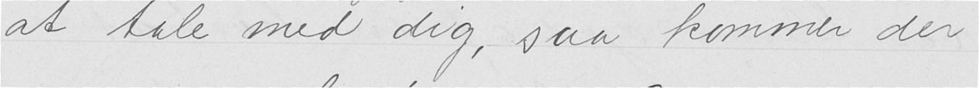at tale med dig, saa kommer der
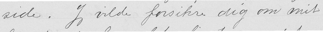side. Jeg vilde forsikre dig om mit
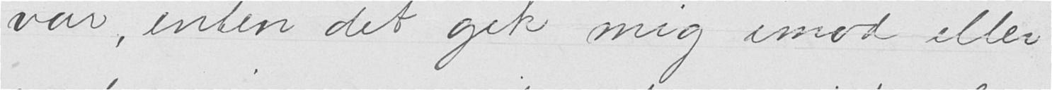var, enten det gik mig imod eller
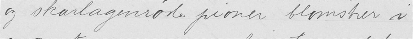og skarlagensrøde pioner blomstrer i
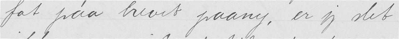fat paa brevet paany, er jeg slet
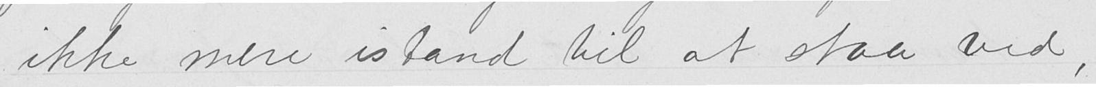ikke mere istand til at staa ved,
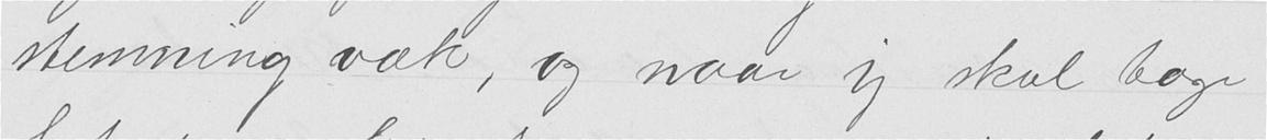stemning væk, og naar jeg skal tage
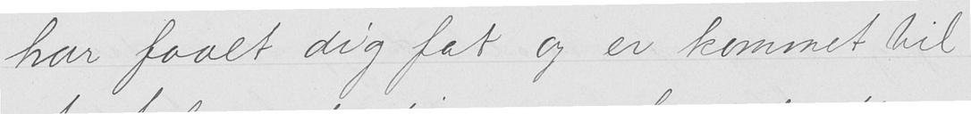har faaet dig fat og er kommet til
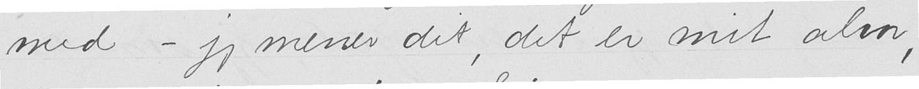med - jeg mener det, det er mit alvor,
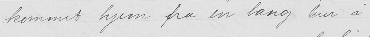kommet hjem fra en lang tur i
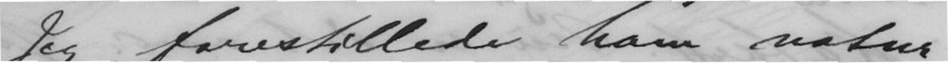Jeg forestillede ham natur-
